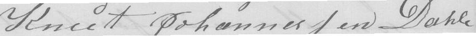Knut Johannessen Dahle
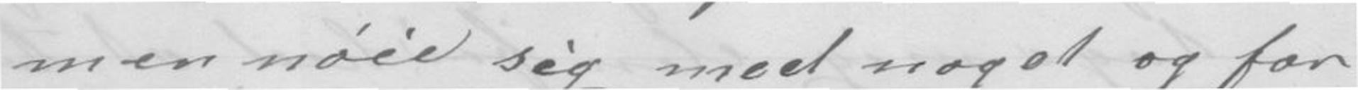men nøie sig med noget og for
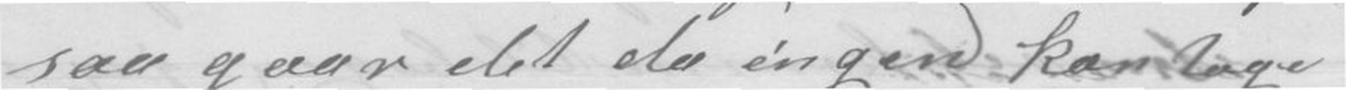saa gaar det da ingen kan tage
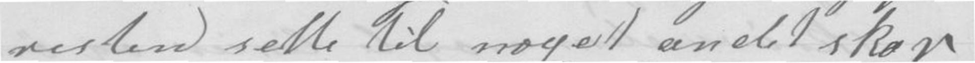resten sette til noget andet skap
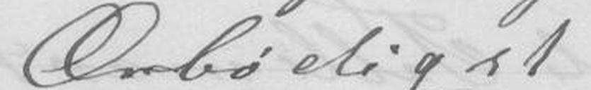Ærbødigst
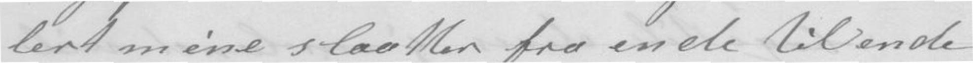lert mine slaatter fra ende til ende
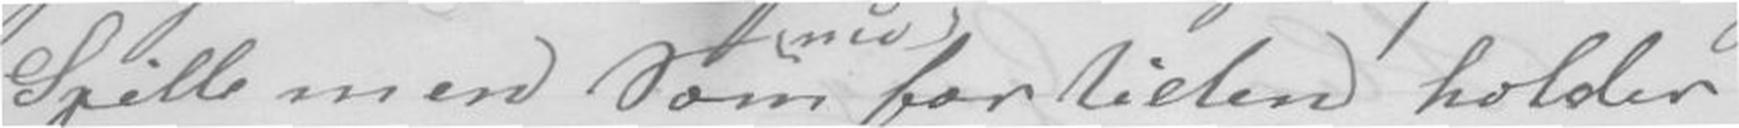Spillemen som for tiden holder
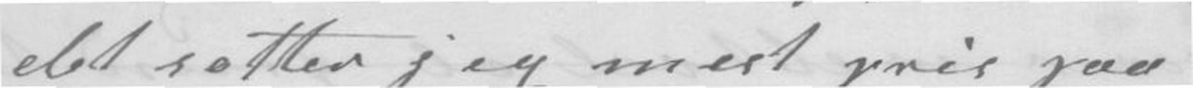det sætter jeg mest pris paa
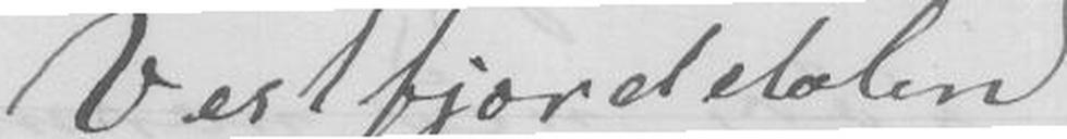Vestfjorddalen
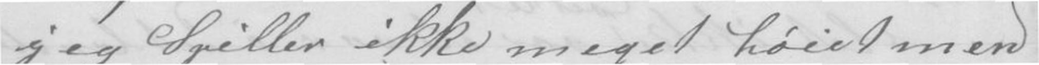jeg Spiller ikke meget høiet men
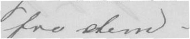fra dem
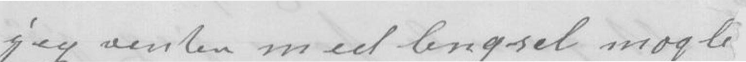jeg venter med lengsel nogle
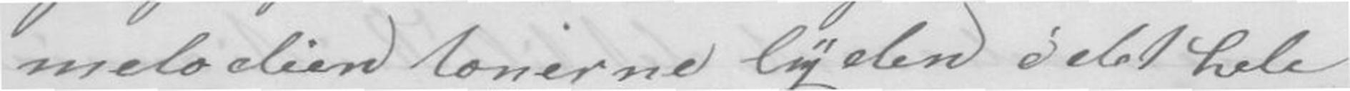melodien tonerne lyden i det hele
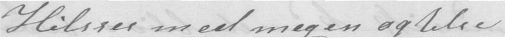Hilsses med megen agtelse
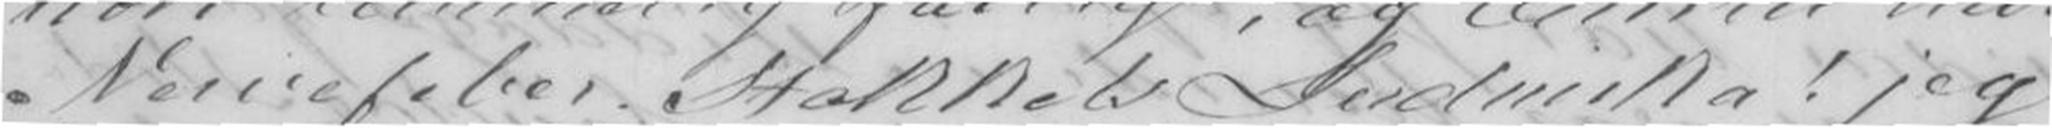Nervefeber. Stakkels Ludniske! jeg
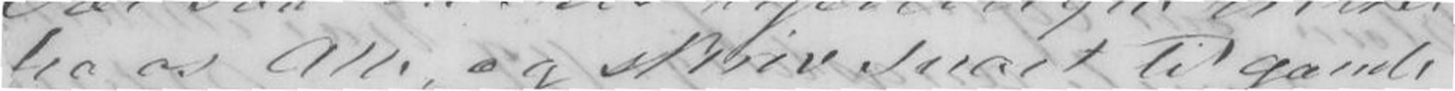ra os Alle, og skriv snart til gamle
